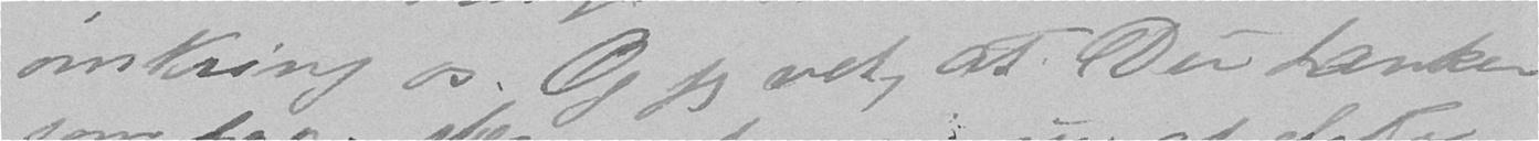omkring os. Og jeg vet, at Du tænker
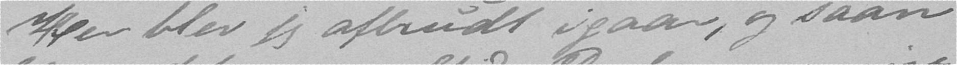Her blev jeg afbrudt igaar, og saan
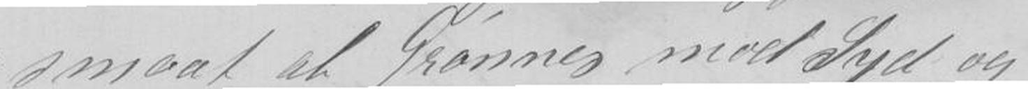smaat at Grønnes mod Syd og
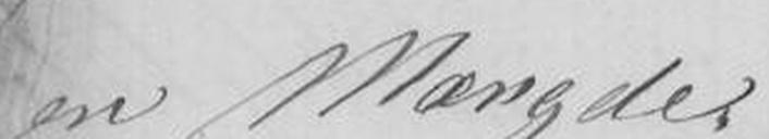en Mængde.
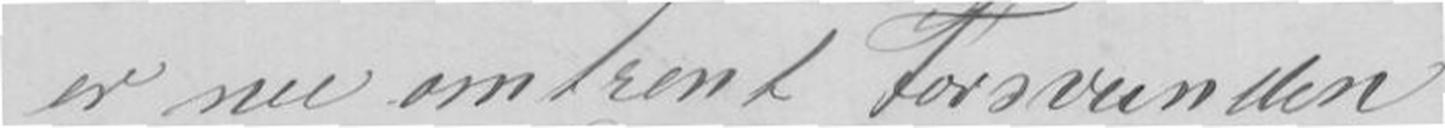er nu omtrent Forsvunden
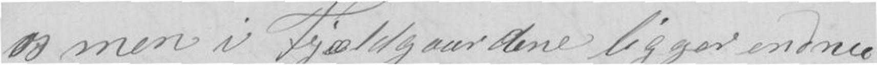os men i Fjældgaardene ligger endnu
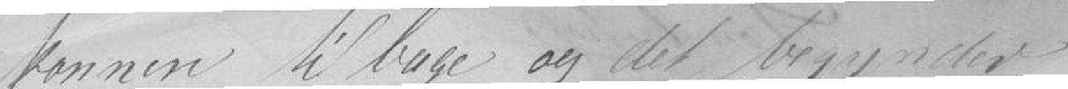kommen tilbage og det begynder
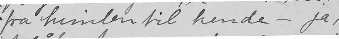fra himlen til hende - ja,
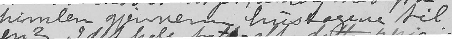himlen gjennem hustagene til
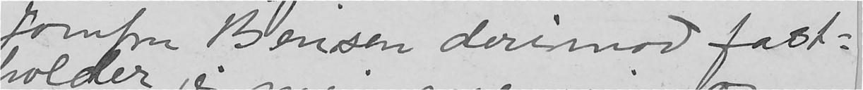Jomfru Bensen derimod fast-
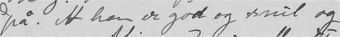på. At han er god og snil og
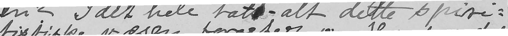en? I det hele tat - alt dette spiri-
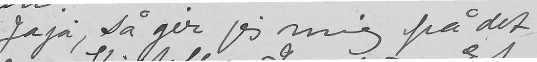Jaja, så gir jeg mig på det
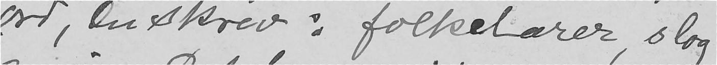ord, Du skrev: folkelærer, slog
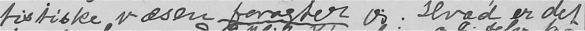tistiske væsen foragter jeg. Hvad er det
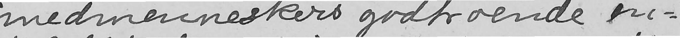medmenneskers godtroende en-
